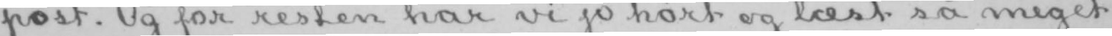post. Og for resten har vi jo hørt og læst så meget
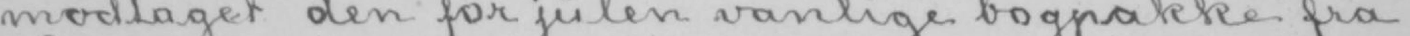modtaget den for julen vanlige bogpakke fra
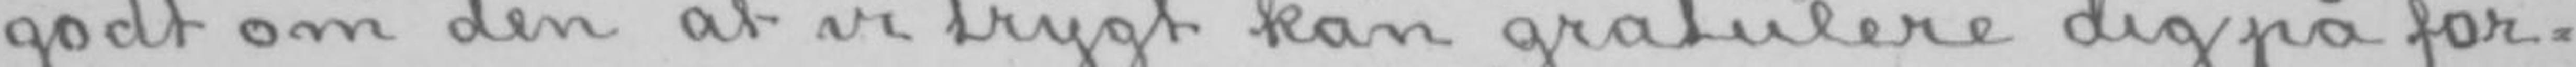godt om den at vi trygt kan gratulere dig på for-
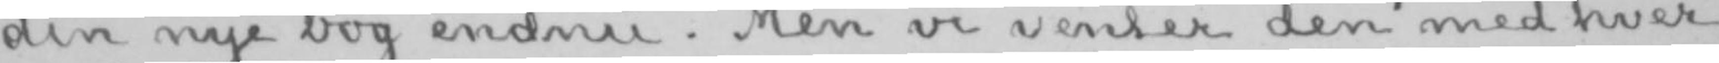din nye bog endnu. Men vi venter den med hver
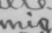mig
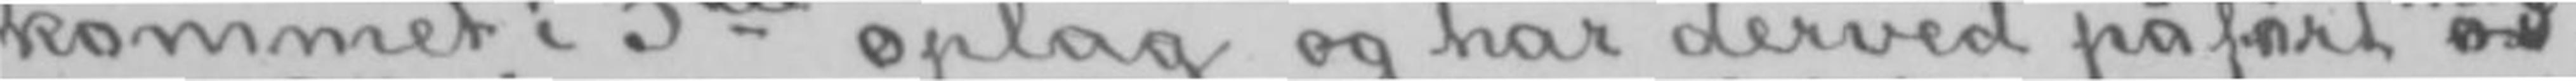kommet i 3die oplag og har derved påført os
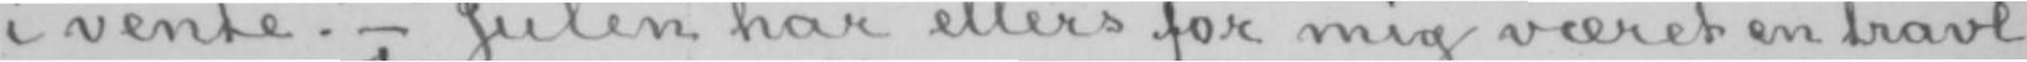i vente.- Julen har ellers for mig været en travl
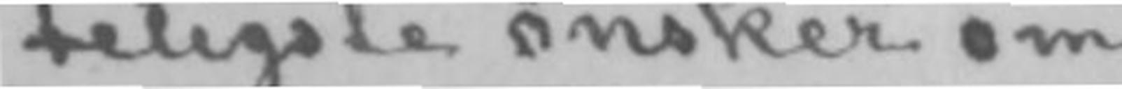teligste ønsker om Bortklippet tekst.
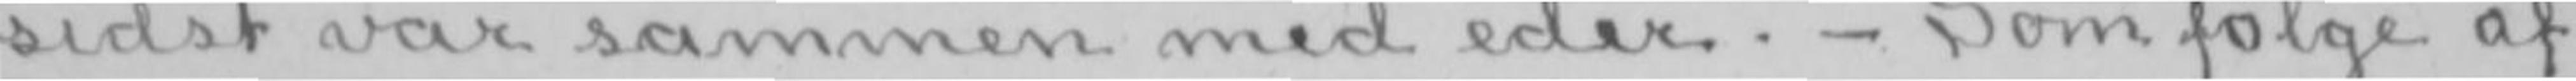sidst var sammen med eder.- Som følge af
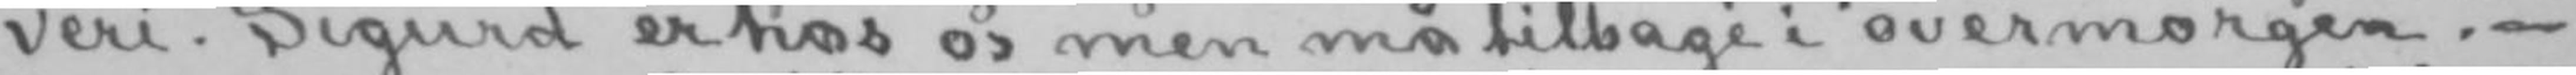veri. Sigurd er hos os men må tilbage i overmorgen.-
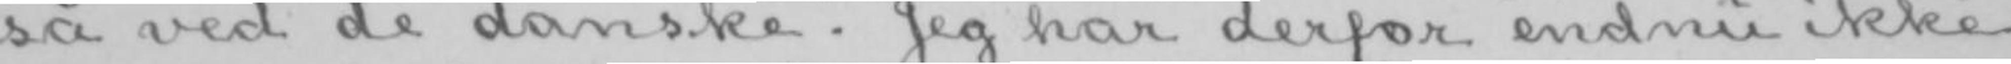så ved de danske. Jeg har derfor endnu ikke
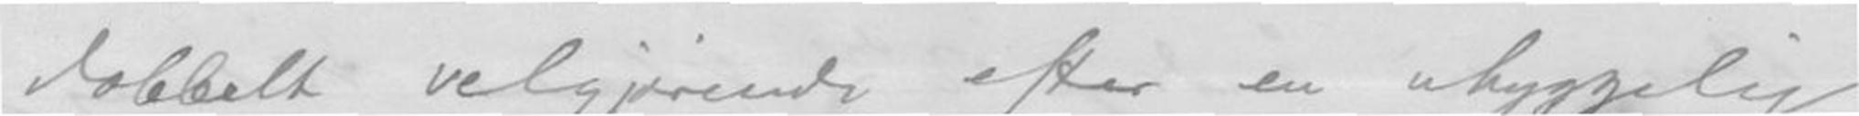dobbelt velgjørende efter en uhyggelig
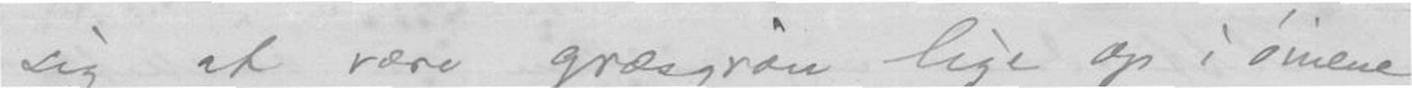sig at være græsgrøn lige op i øinene
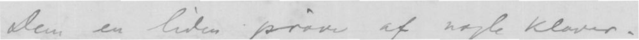Dem en liden prøve af nogle klaverstykker,
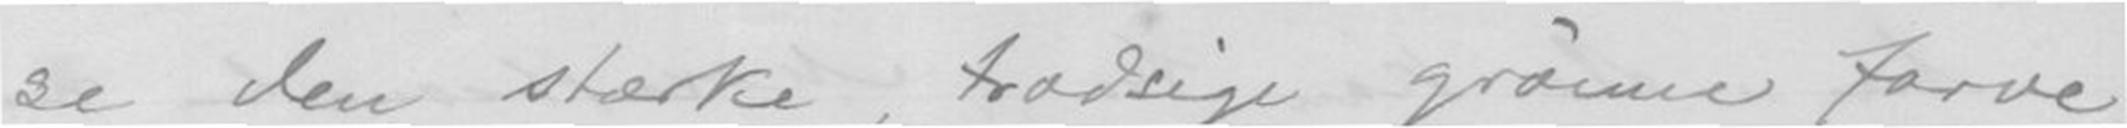se den stærke, trodsige grønne farve
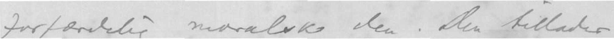forfærdelig moralske den. Den tillader
